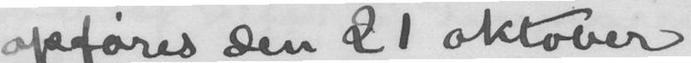opføres den 21 oktober.
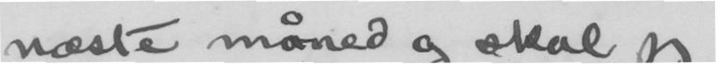næste nåned og skal jeg
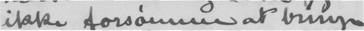ikke forsømme at bringe
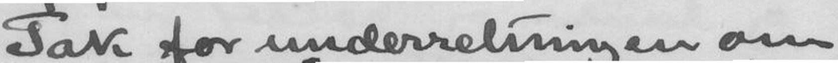Tak for underretningen om
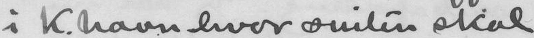i K.havn hvor suiten skal
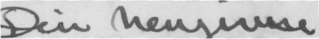Din hengivne
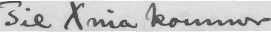Til Xnia kommer
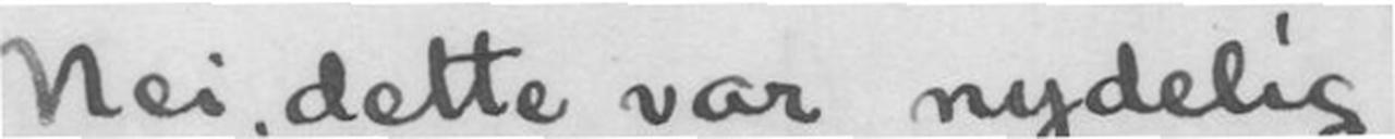Nei, dette var nydelig!
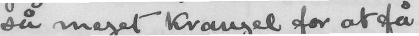så meget krangel for at få
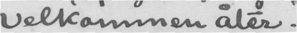Velkommen åter.
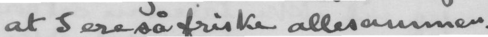at I ere så friske allesammen.
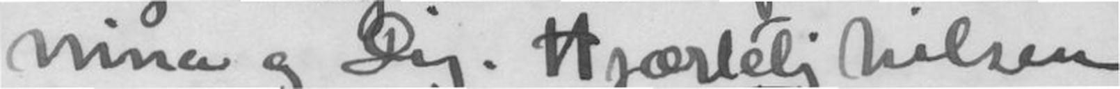Nina og Dig. Hjærtelig hilsen
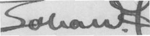Johan H.
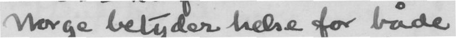Norge betyder helse for både
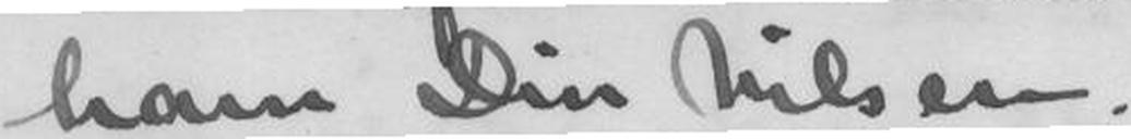ham Din hilsen.
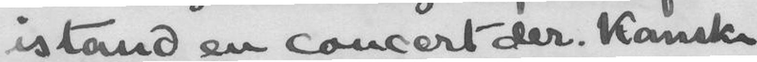istand en concert der. Kanske
